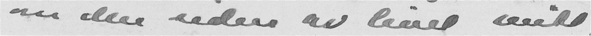om den siden av livet mitt,
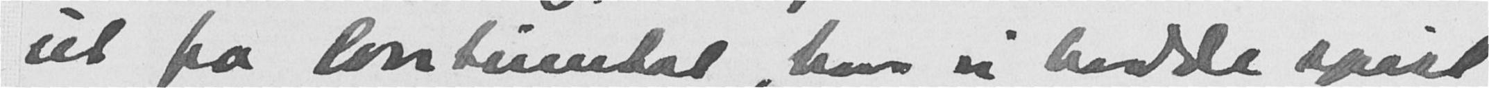ut fra Aontuntat, hvor vi hadde spist
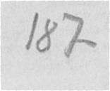187
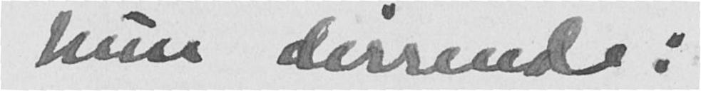hun dirrende:
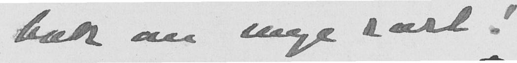bok om mye rart!
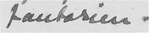fantasien.
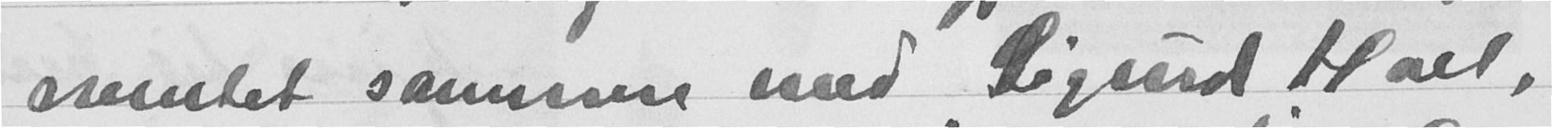mentet sammen med Sigurd Hoel,
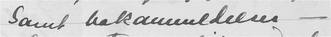Samt bokanmeldelser -
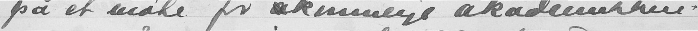på et møte for kvinnelige akademikkere.
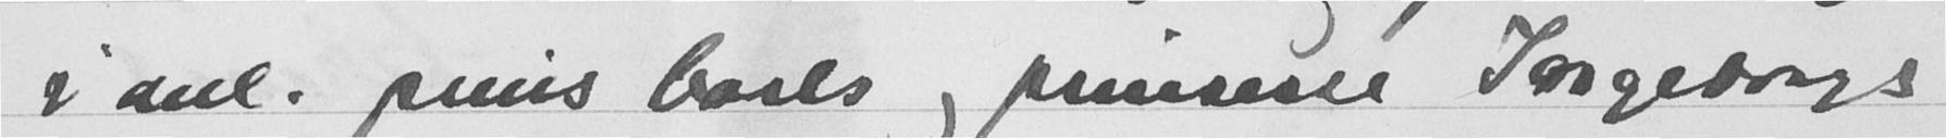i anl. prins Karls og prinsesse Ingeborgs
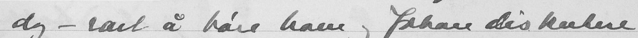dag - rart å høre ham og Johan diskutere
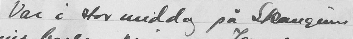Var i stor middag på Skaugum
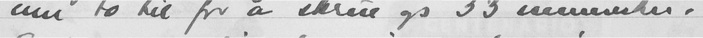enn to til for å skrue op 33 mennesker.
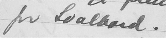for Svalbard.
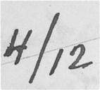4/12
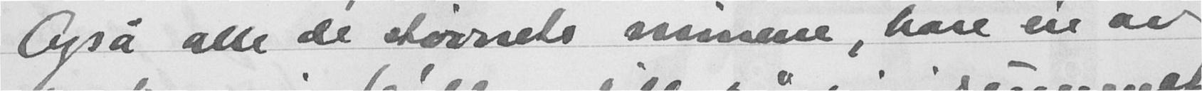Også alle de støvete minnene, bare en av
